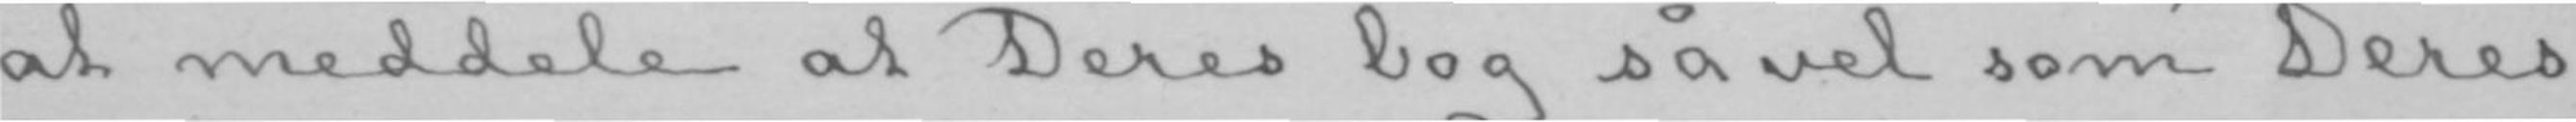at meddele at Deres bog såvel som Deres
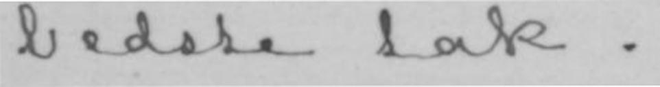bedste tak.
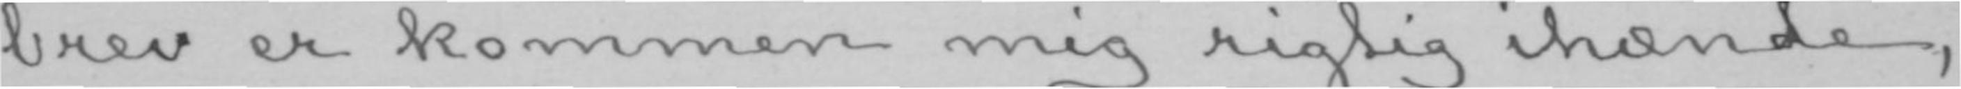brev er kommen mig rigtig ihænde,
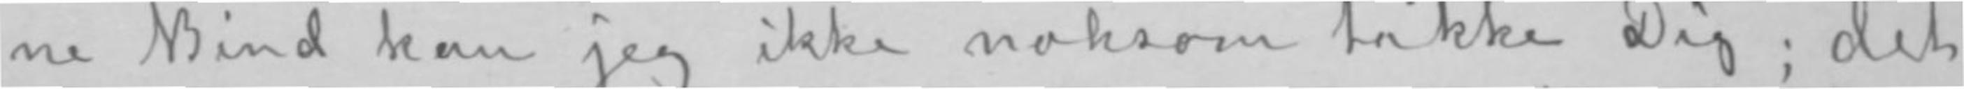ne Bind kan jeg ikke noksom takke Dig; det
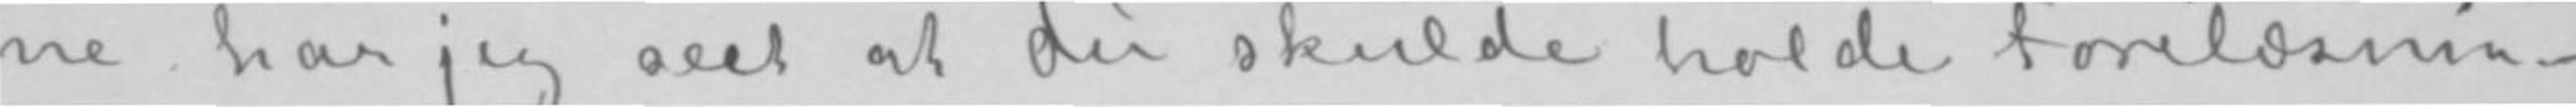ne har jeg seet at Du skulde holde Forelæsnin-
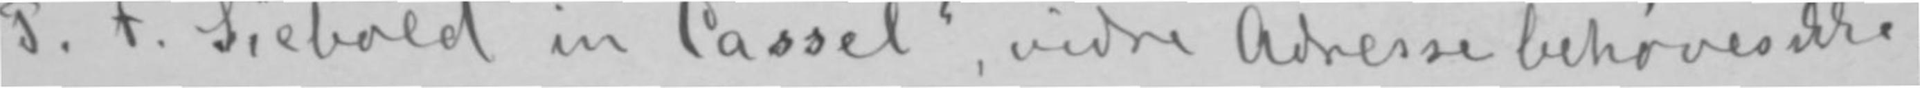«P. F. Siebold in Cassel», vidre Adresse behøves ikke;
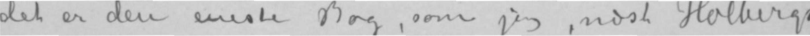det er den eneste Bog, som jeg, næst Holbergs
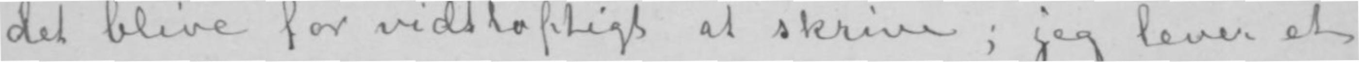det blive for vidtløftigt at skrive; jeg lever et
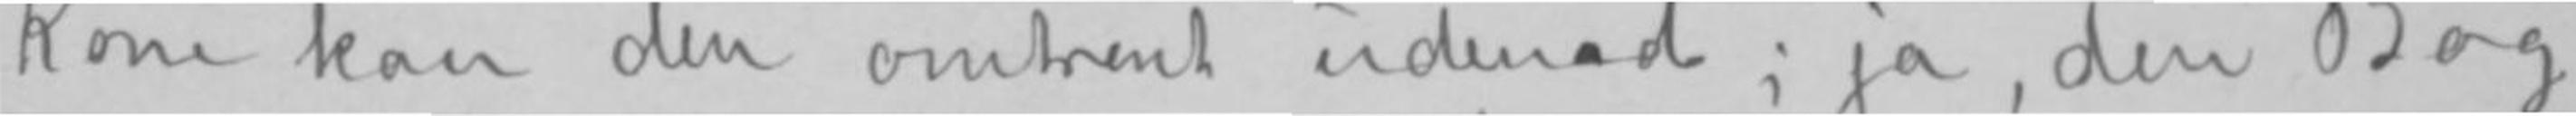Kone kan den omtrent udenad; ja, den Bog
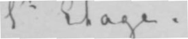1te Etage.»
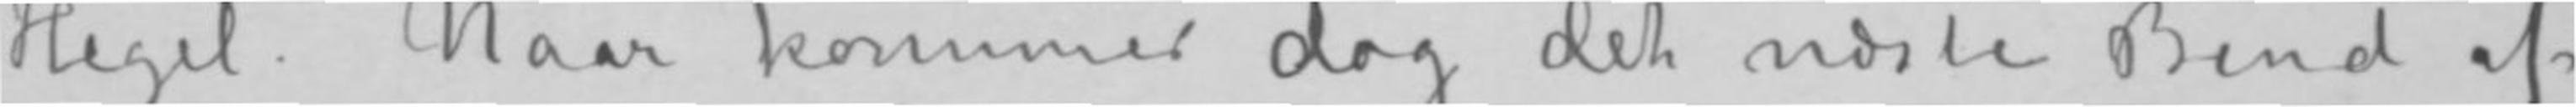Hegel. Naar kommer dog det næste Bind af
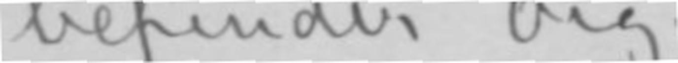befinder Dig
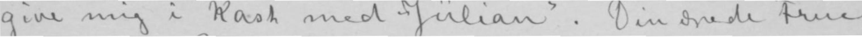give mig i Kast med «Julian». Din ærede Frue
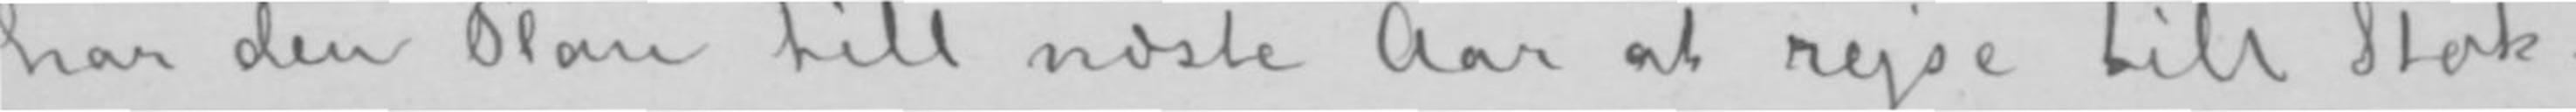har den Plan till næste Aar at rejse till Stok-
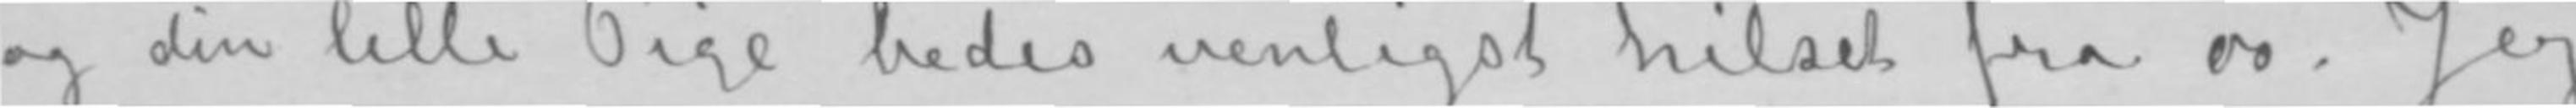og din lille Pige bedes venligst hilset fra os. Jeg
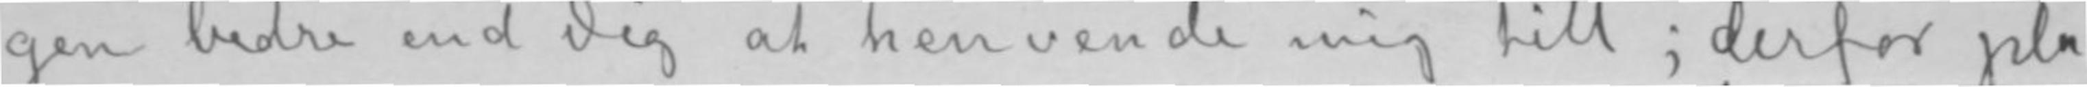gen bedre end Dig at henvende mig till; derfor pla-
